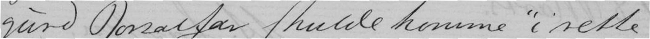gurd Jorsalfar skulde komme "i rette
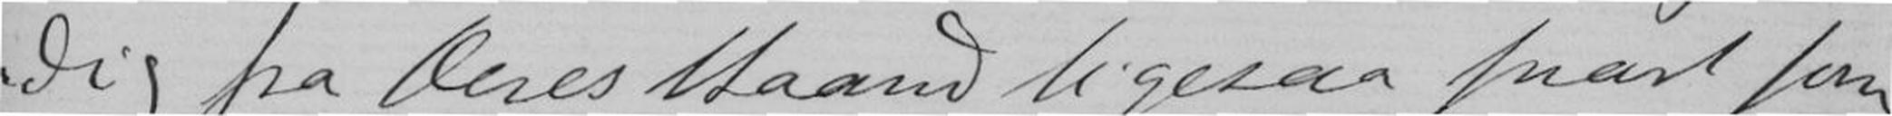dig fra Deres Haand ligesaa snart som
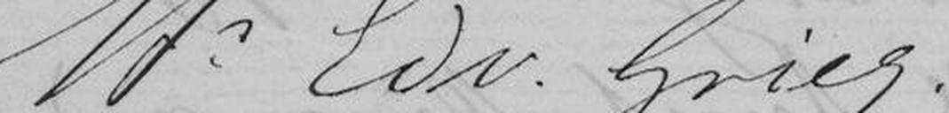Mr Edv. Grieg !
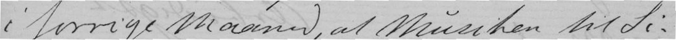i forrige maaned, at Musiken til Si-
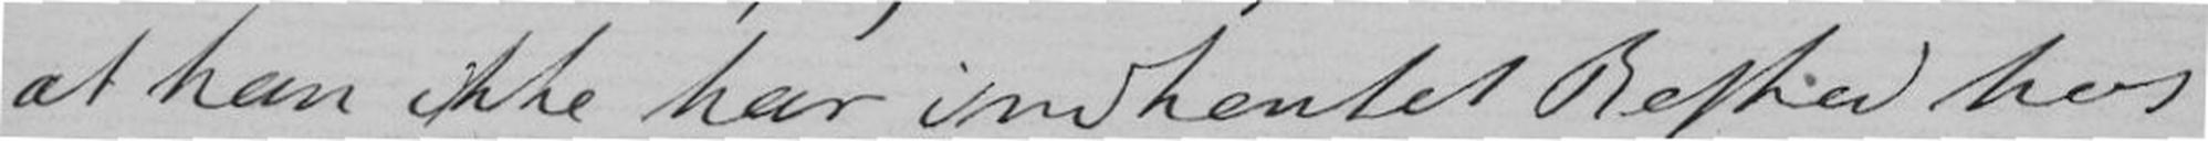at han ikke har inhentet Besked hos
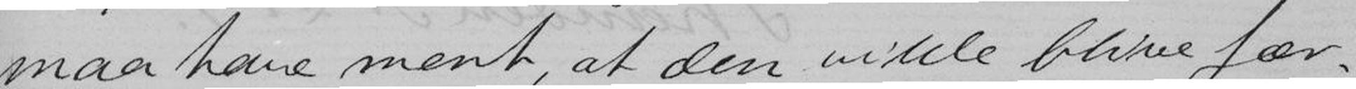maa have ment, at den vilde blive fær-
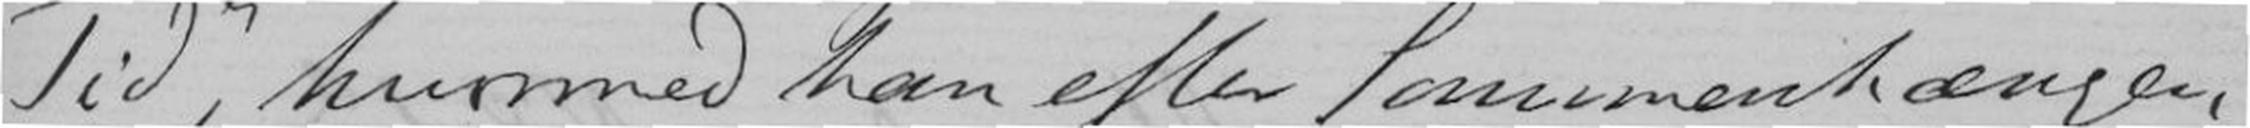Tid", hermed han efter Sammen..ængen,
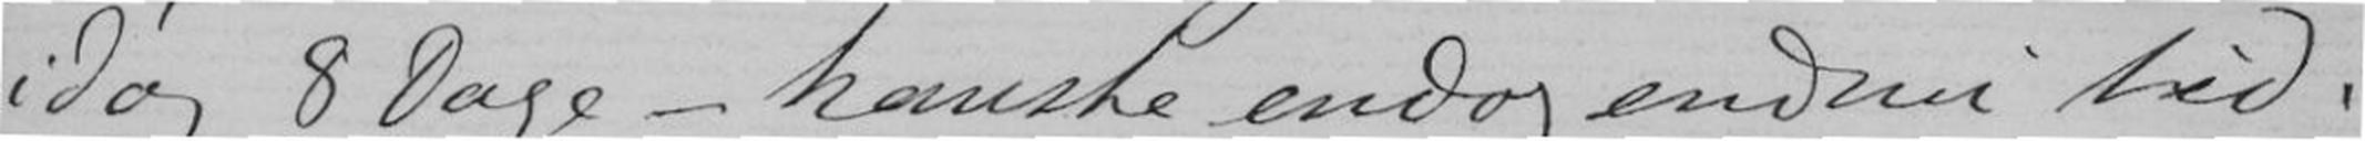idag 8 Dage - kanske endog endnu tid-
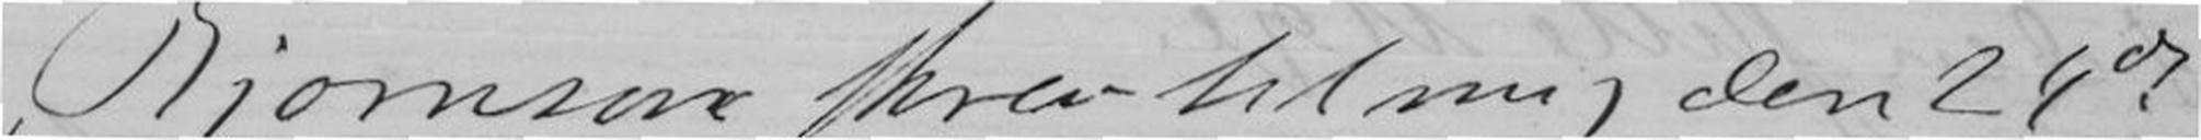Bjørnson skrev til mig den 24e
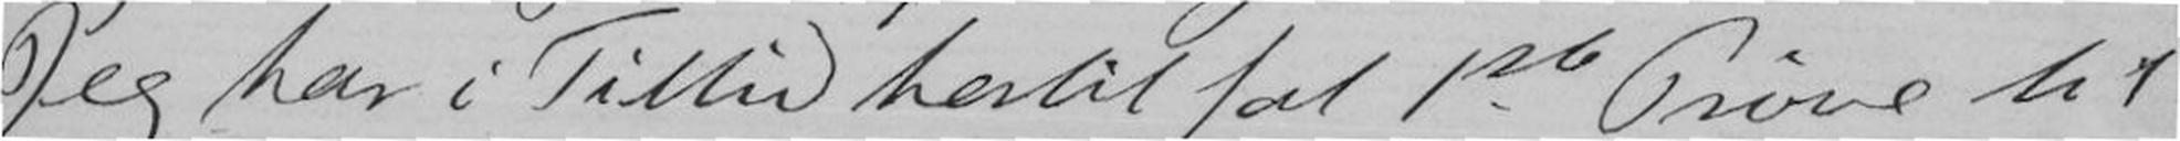Jeg har i Tillid hertil sat 1ste Prøve til
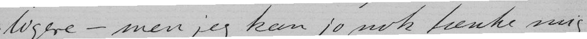ligere - men jeg kan jo nok tænke mig
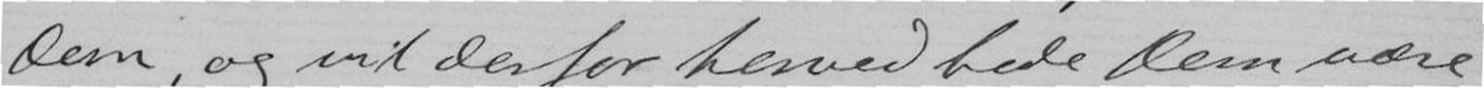Dem, og vil derfor herved bede Dem være
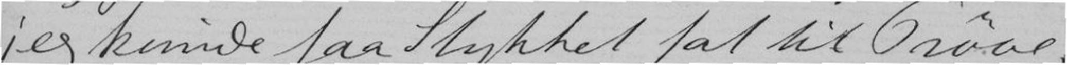jeg kunde faa Stykket fat til Prøve.
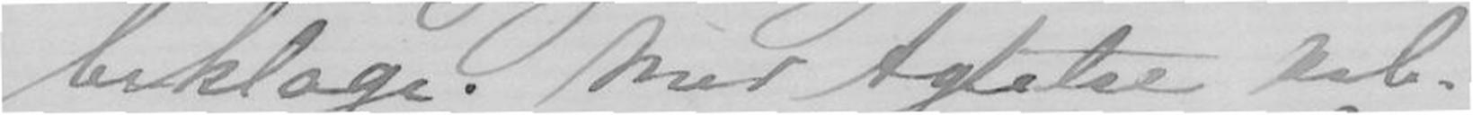beklage. Med Agtelse Ærb.
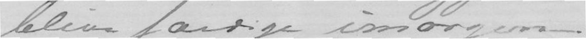blive færdige imorgen
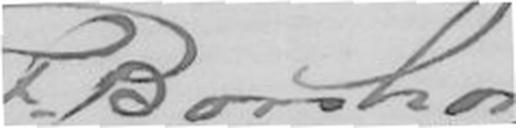F. Borchorst.
